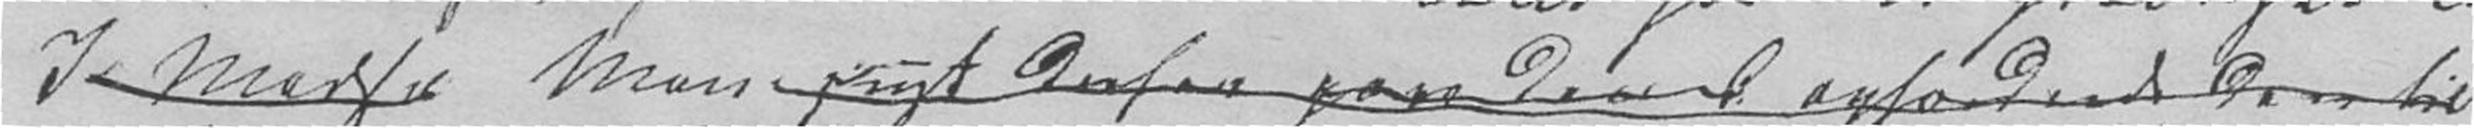Men
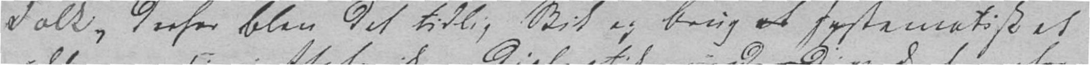Folk, derfor blev det tidlig Skik og Brug systematisk at
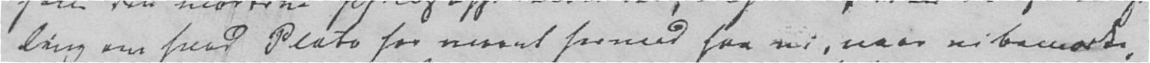ling om hvad Plato har meent hermed faa vi, naar vi bemærke,
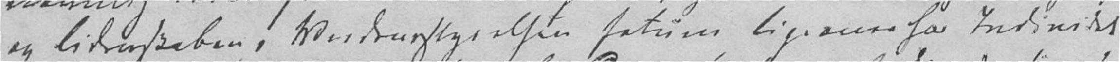og Lidenskaben, Verdensstyrelsen fatum ligeoverfor Individet
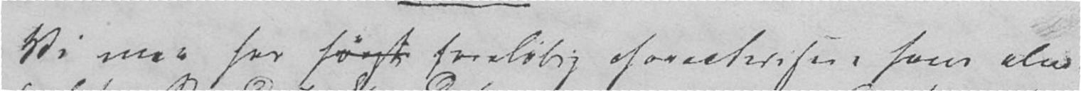Vi maa her foreløbig characterisere hans alm:
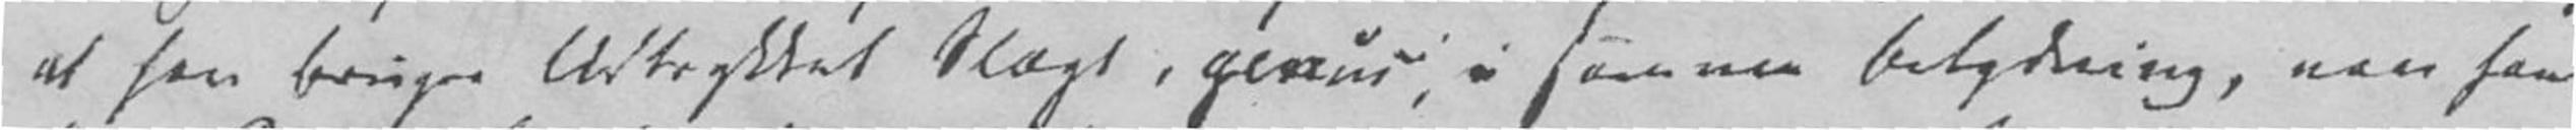at han bruger Udtrykket Slægt, genus, i samme Betydning, naar han
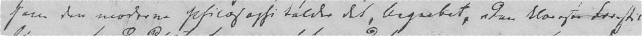som den moderne Philosophi kalder det Begrebet. Den klareste Forestil-
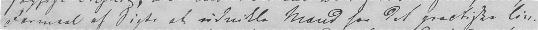Formaal af Sigte at udvikle Mænd for det practiske Liv.
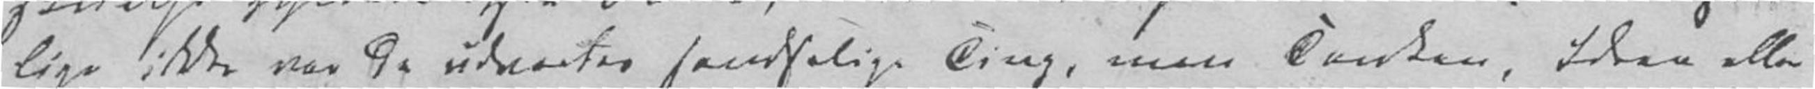lige ikke var de udvortes sandselige Ting, men Tanken, Ideen eller
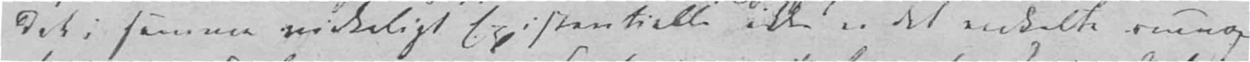det i samme virkeligt Existentielle ikke er det enkelte sin og
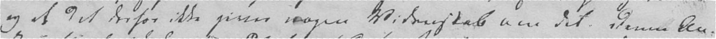og at det derfor ikke gives nogen Videnskab om det. Denne An-
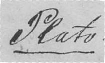Plato
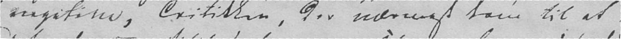negative, Critikken, der nærmest kom til at
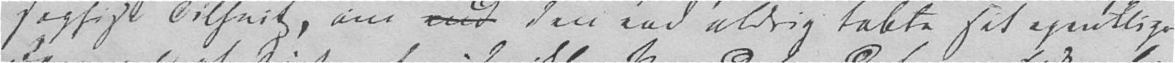sophisk Tilsnit, om den end aldrig tabte sit egentlige
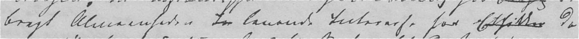bragt Almeenheden levende Interesse for de
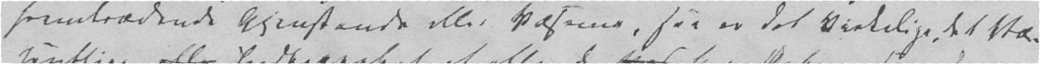fremtrædende Gjenstande eller Væsener, saa er det Virkelige, det Væ-
